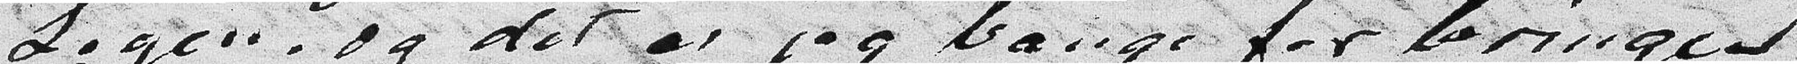Legen, og er jeg bange for bringer
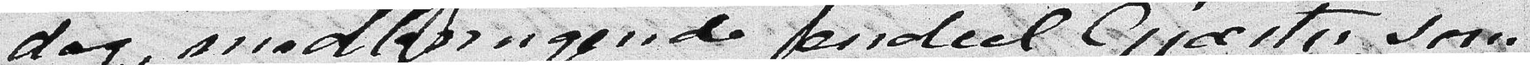dag, medbringende endeel Gjæster, som
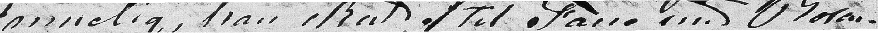muelig, han skulde til Fana med Rosen-
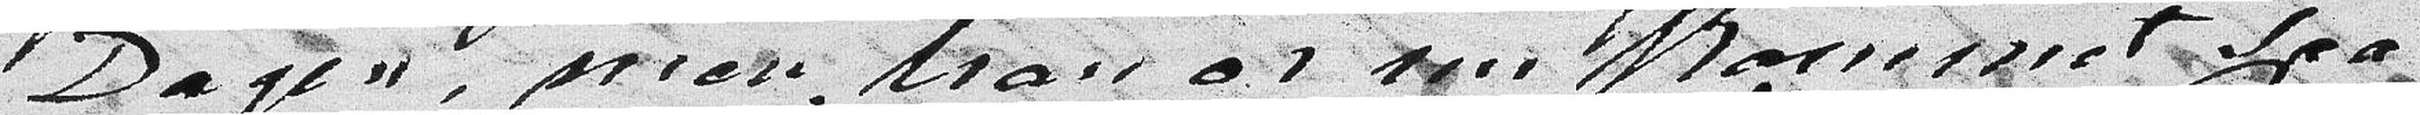Dagen, men han er nu kommet saa
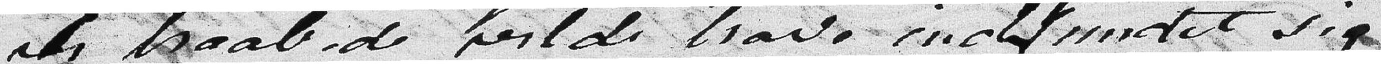vi haabede vilde have indfundet sig
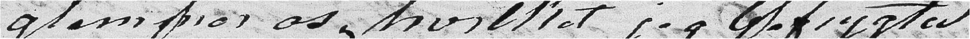glemmer os, hvilket jeg befrygter
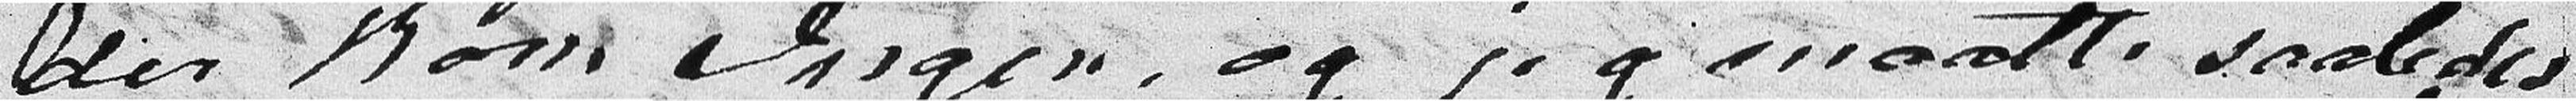der kom Ingen, og jeg maatte saaledes
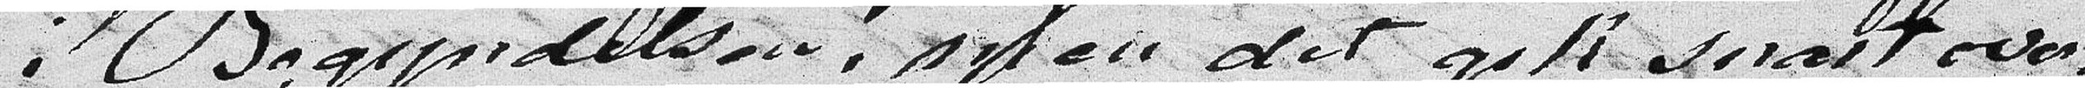i Begyndelsen, men det gik snart over,
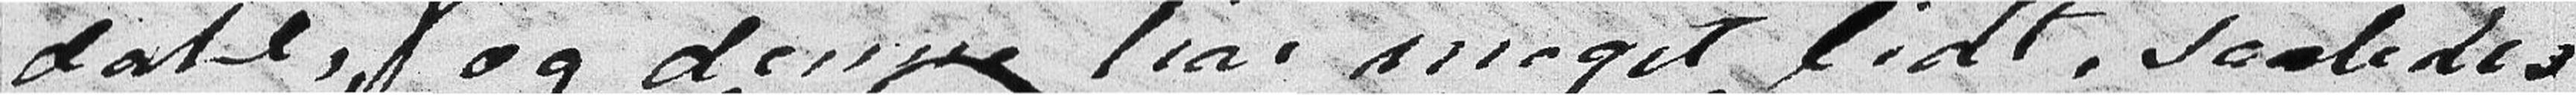dahl, og denne har meget lidt, saaledes
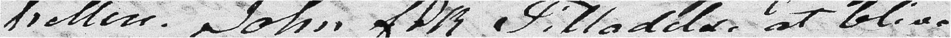hellere. John fik Tiladelse at blive
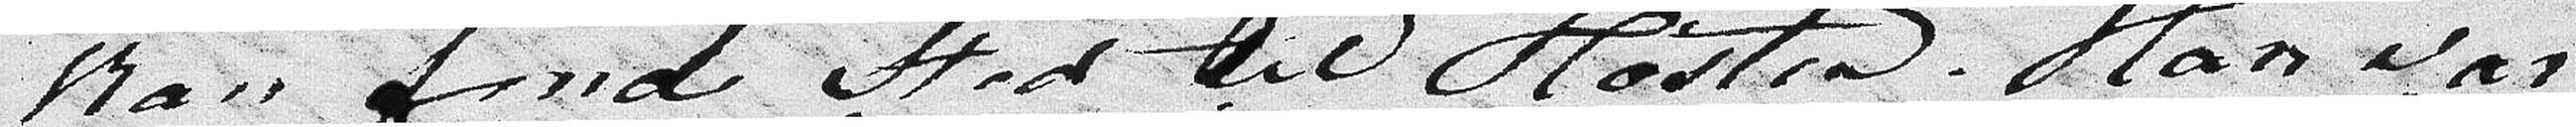kan finde Sted til Høsten. Han var
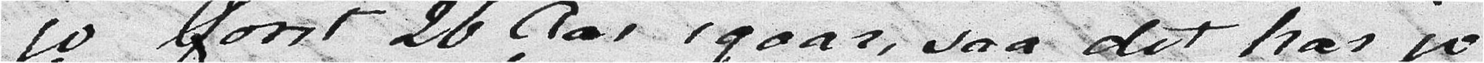jo først 26 Aar igaar, saa det har jo
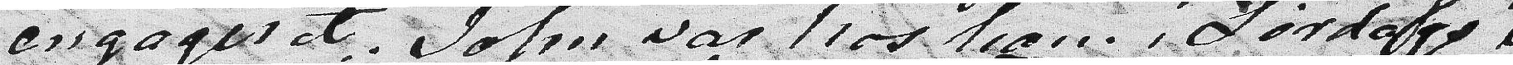engageret. John var hos ham i Lørdags
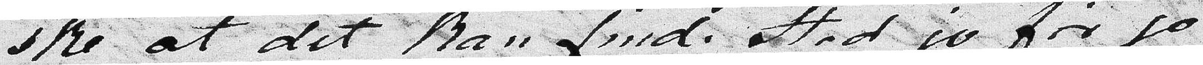ske at det kan finde Sted jo før jo
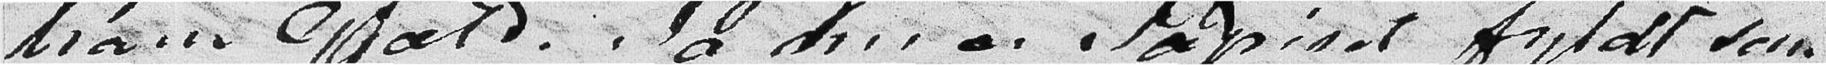ham Gjæld. Ja nu er Papiret fyldt som
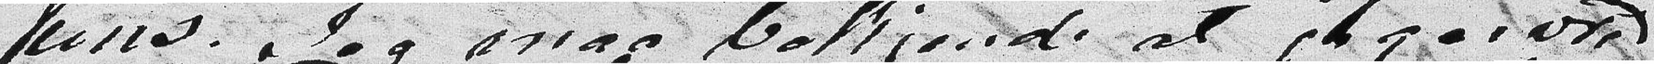uns. Jeg maa bekjnde at jeg er vred
